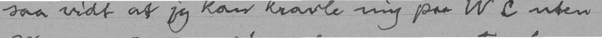saa vidt at jeg kan kravle mig paa W C uten
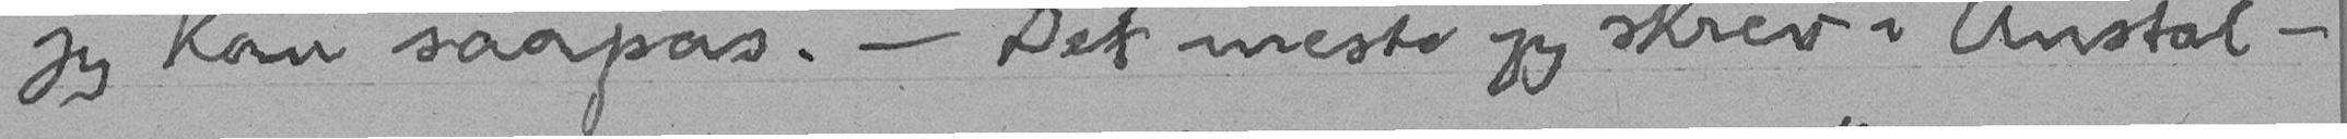jeg kan saapas. - Det meste jeg skrev i Anstal -
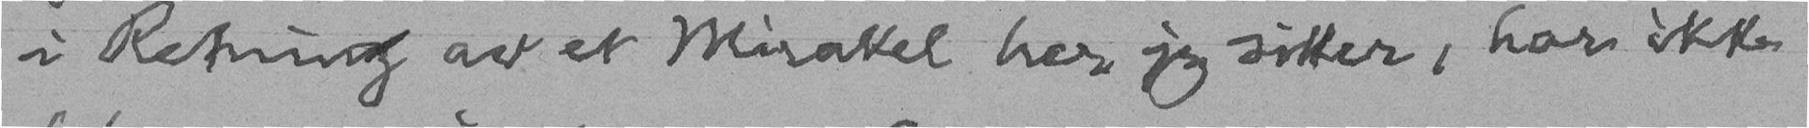i Retning av et Mirakel her jeg sitter, har ikke
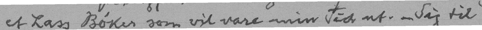et Lass Bøker som vil vare min Tid ut. - Sig til
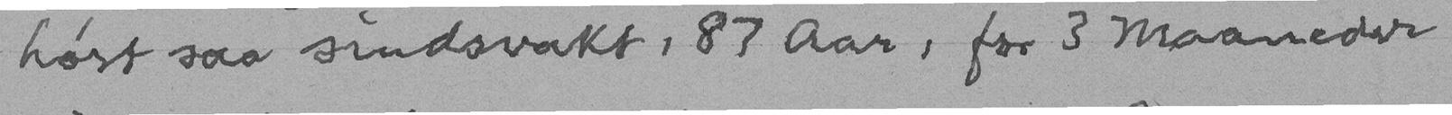hørt saa sindsvakt, 87 Aar, for 3 Maaneder
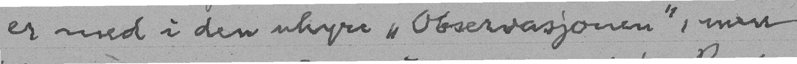er med i den uhyre "Observasjonen", men
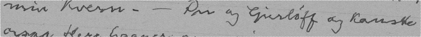min Kvern. - Du og Gierløff og kanske
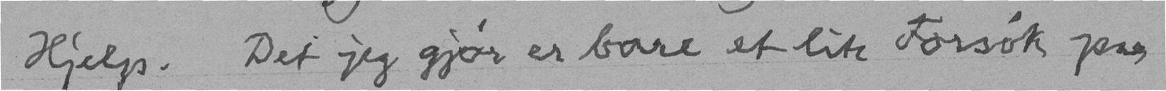Hjelp. Det jeg gjør er bare et lite Forsøk paa
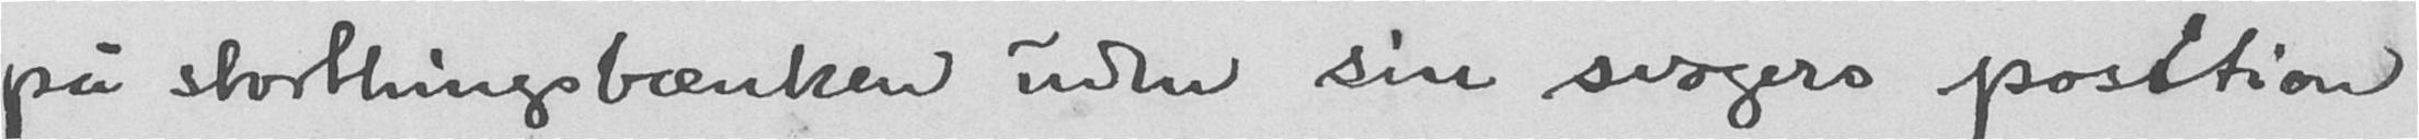på storthingsbænken uden sin svogers position
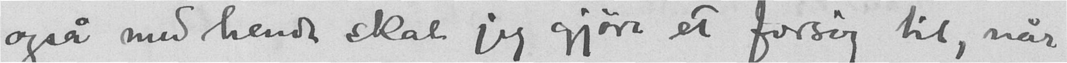også med hende skal jeg gjøre et forsøg til, når
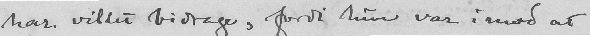har villet bidrage, fordi hun var imod at
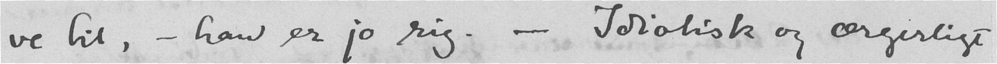ve til- han er jo rig. - Idiodisk og ærgerligt
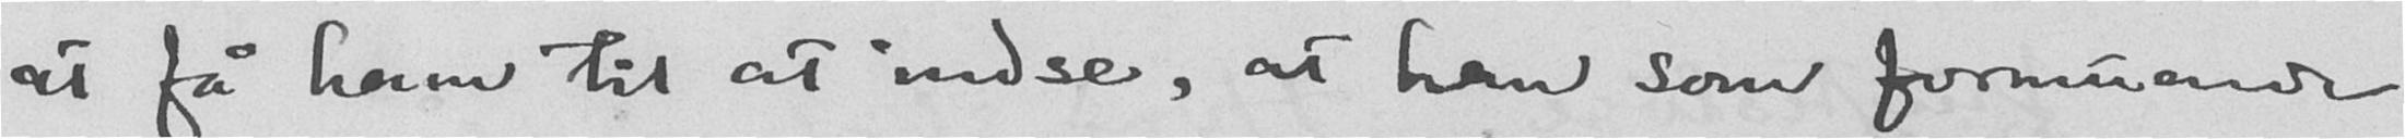at få ham til at indse, at han som formuende
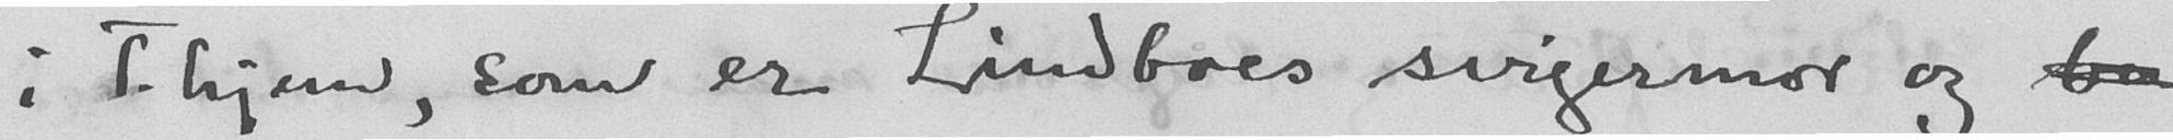i T. hjem, som er Lindboes svigermor og bu
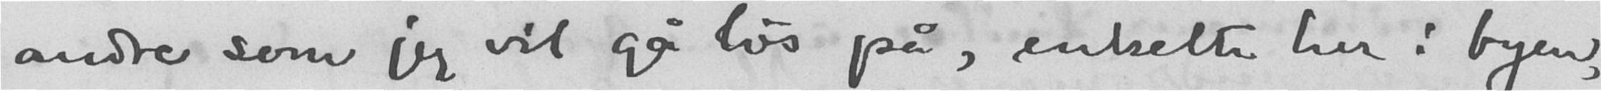andre som jeg vil gå løs på, enkelte her i byen,
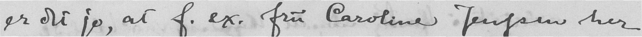er det jo, at f. ex. fru Caroline Jenssen her
